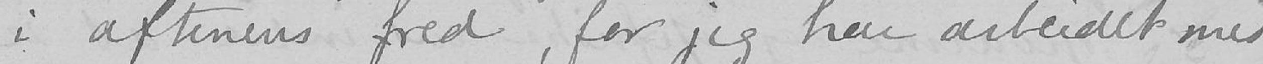i aftenens fred, for jeg har arbeidet med
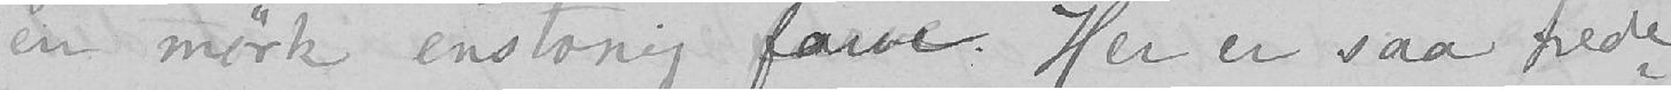en mørk enstonig farve. Her er saa frede-
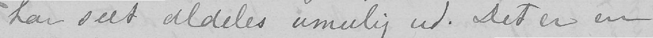har seet aldeles umulig ud. Det er en
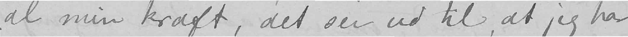al min kraft, det ser ud til, at jeg har
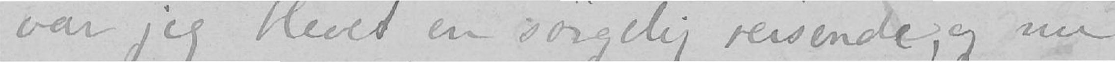var jeg blevet en sørgelig reisende, og nu
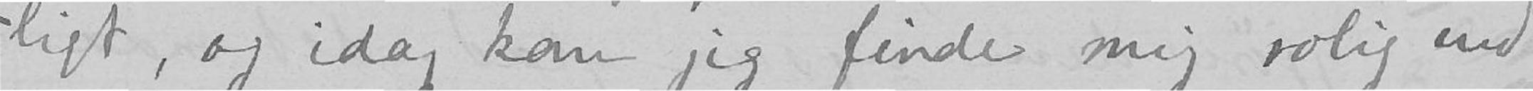ligt, og idag kan jeg finde mig rolig ind
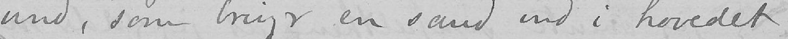vind, som bringer en sand ind i hovedet
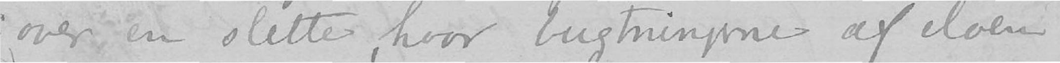over en slette, hvor bugtningerne af elven
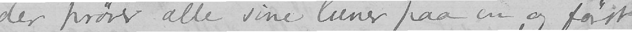der prøver alle sine luner paa en, og først
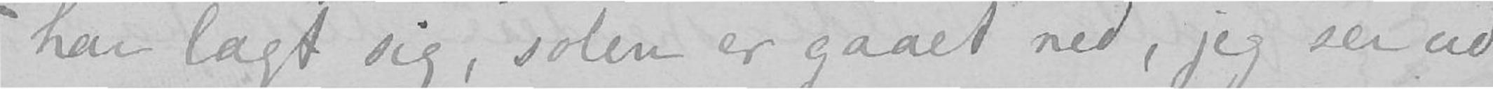har lagt sig, solen er gaaet ned, jeg ser ud
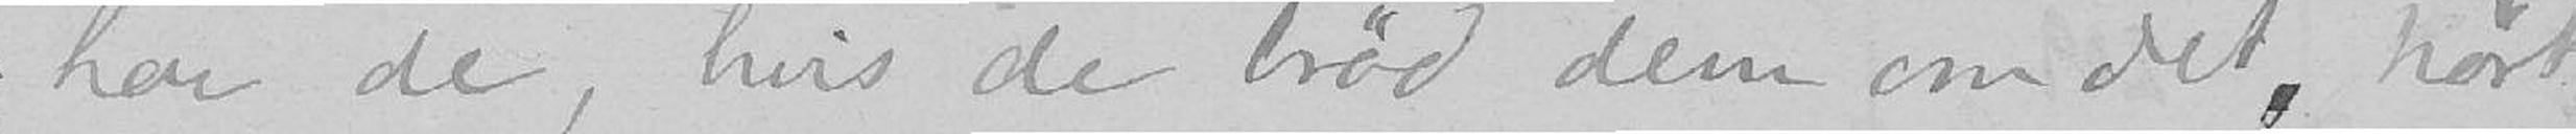har de, hvis de brød dem om det, hørt
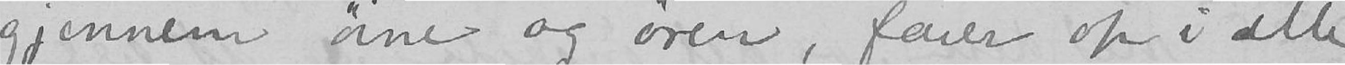gjennem øine og øren, farer op i alle
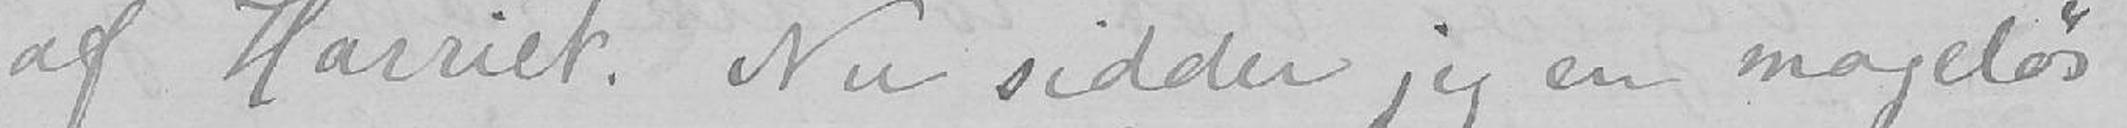af Harriet. Nu sidder jeg en mageløs
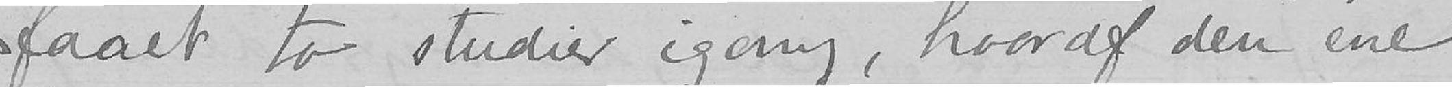faaet to studier igang, hvoraf den ene
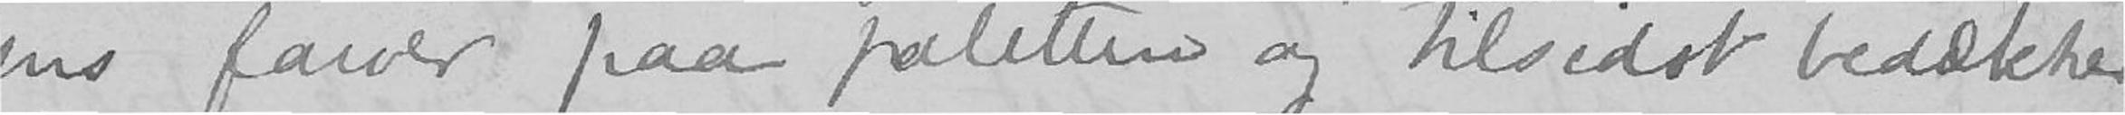ens farver paa paletten og tilsidst bedækker
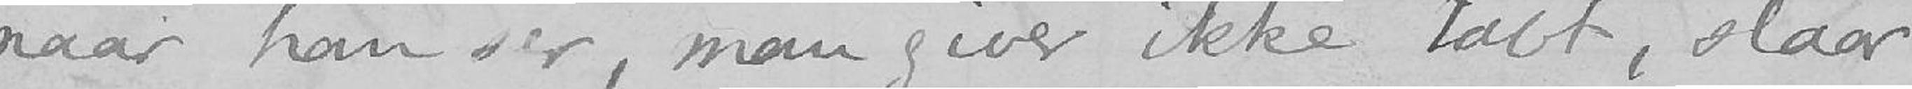naar han ser, man giver ikke tabt, slaar
